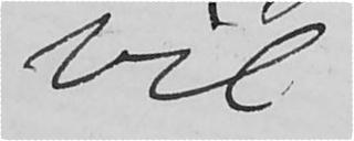vil
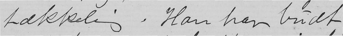tækkelig. Han har budt
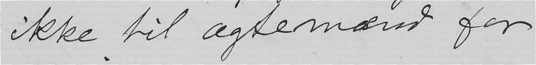ikke til ægtemænd for
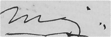mig.
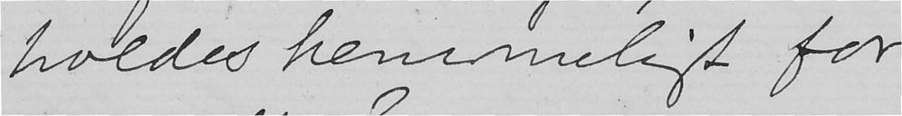holdes hemmeligt for
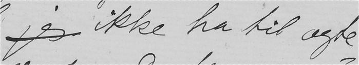jeg ikke ha til ægte-
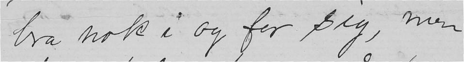bra nok i og for sig, men
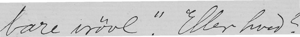bare vrøvl". Eller hvad?
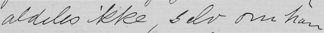aldeles ikke, selv om han
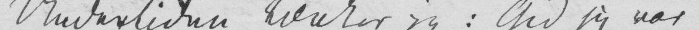Undertiden tænker jeg: Gid jeg var
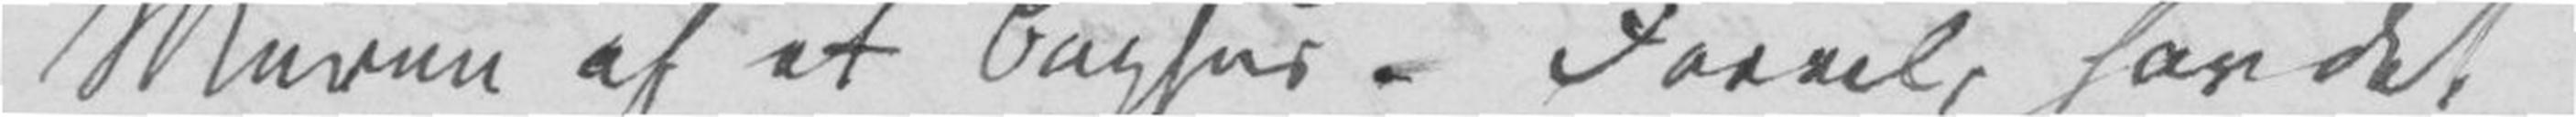Muren af et Baghus. Farvel, hav det
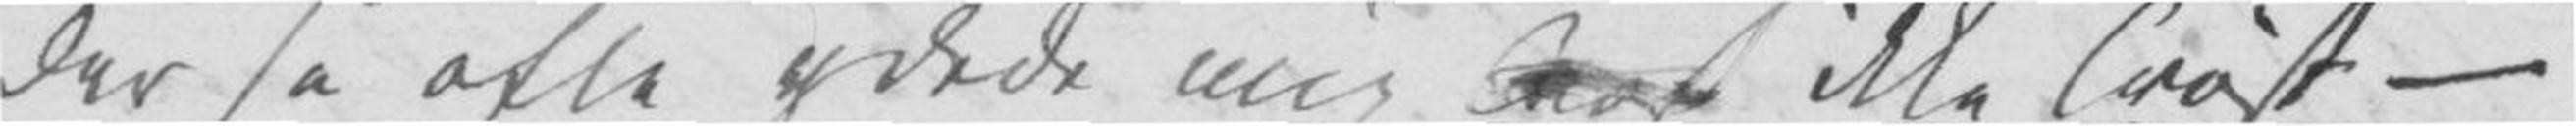der så ofte ydede mig Trøst ikke Trøst –
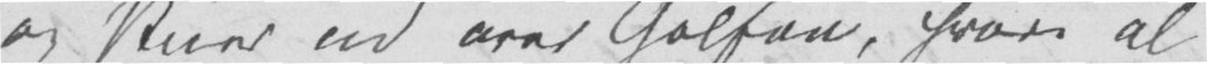og skuer ud over Golfen, hvori al
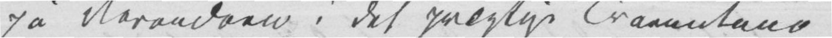på Verandaen i det prægtige Tramontano
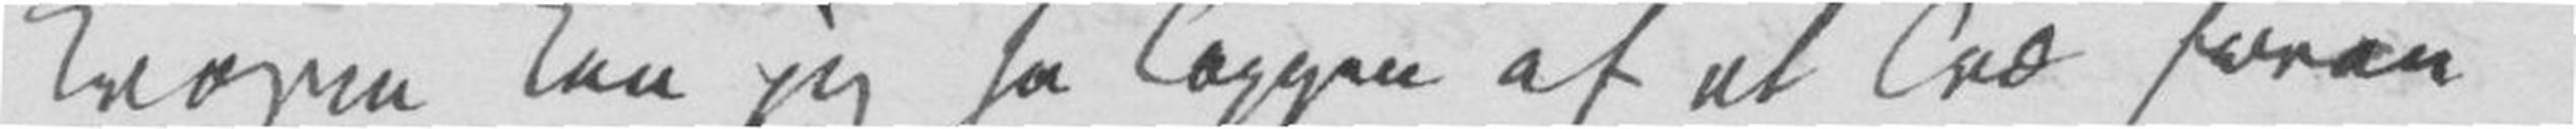i Krogen kan jeg se Toppen af et Træ foran
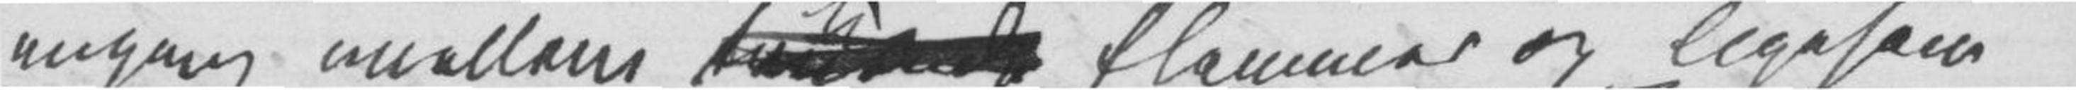engang imellem truende flammer op ligesom
